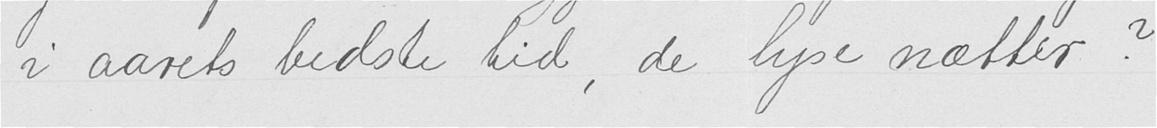i aarets bedste tid, de lyse nætter?
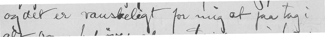og det er vanskeligt for mig at faa tag i
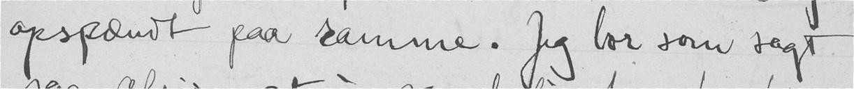opspændt paa samme. Jeg bor som sagt
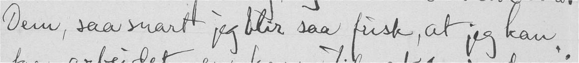Dem, saa snartt jeg blir saa frisk, at jeg kan
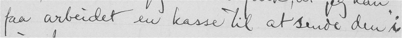faa arbeidet en kasse til at sende den i
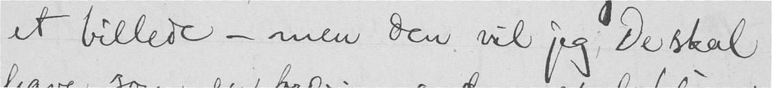et billede - men den vil jeg, De skal
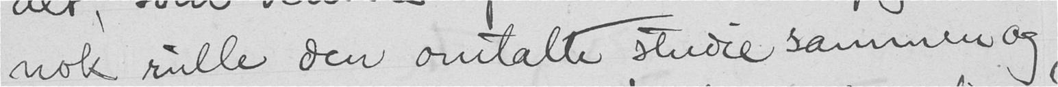nok rulle den omtalte stude sammen og
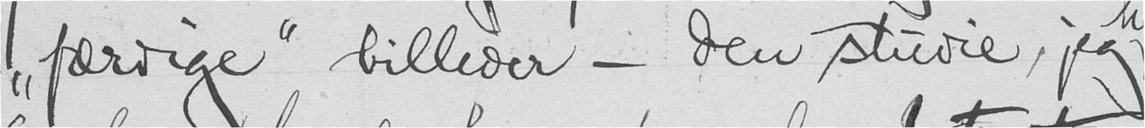"færdige" billeder - den studie, jeg
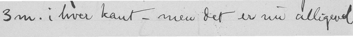3 m. i hver kant - men det er nu alligevel
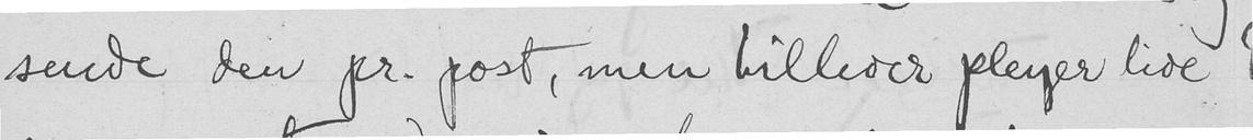sende den pr. post, men billeder pleyer lide
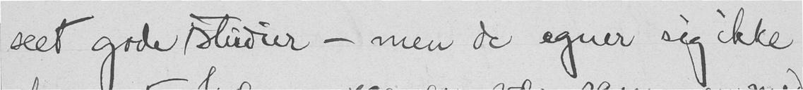seet gode studier - men de egner sig ikke
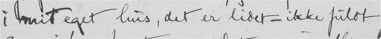i mit eget hus, det er lidet - ikke fuldt
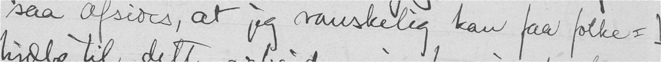saa afsides, at jeg vanskelig kan faa folke-
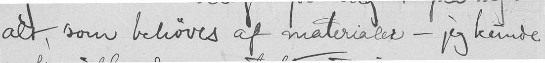alt, som behøves af materialer - jeg kunde
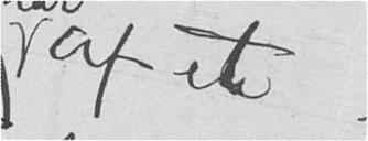af et
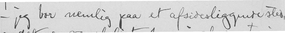- jeg bor nemlig paa et afsidesliggende sted,
